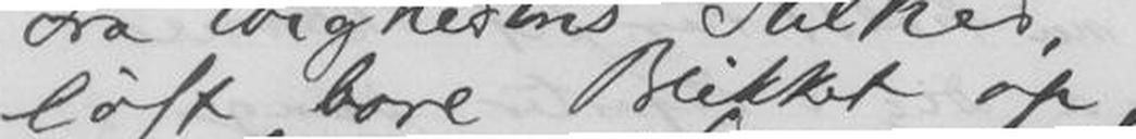løft bare Blikket op
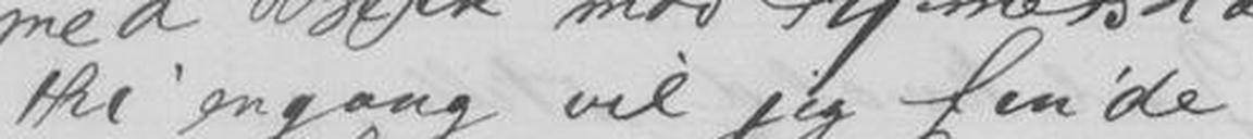Her engang vil jeg finde
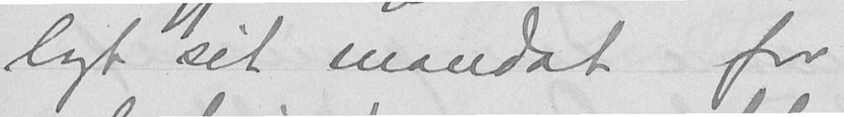lagt sit mandat for
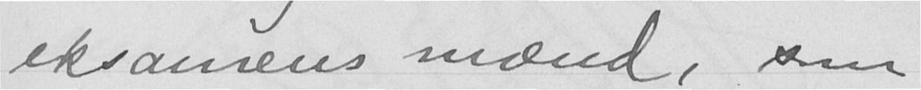eksamens mænd, som
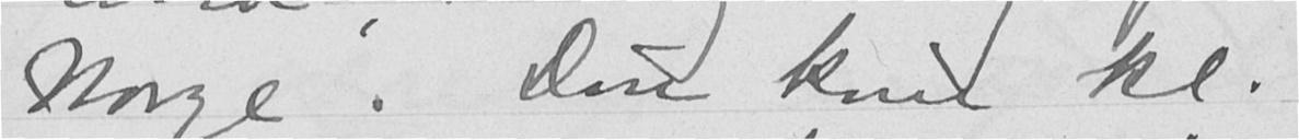Norge. Don kom kl.
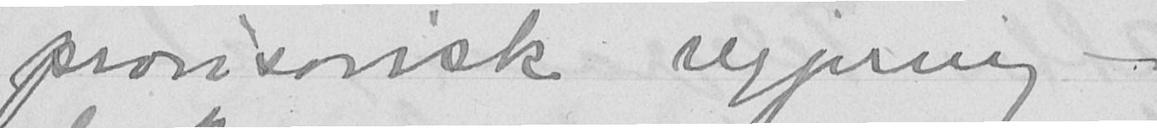provisorisk regjering -
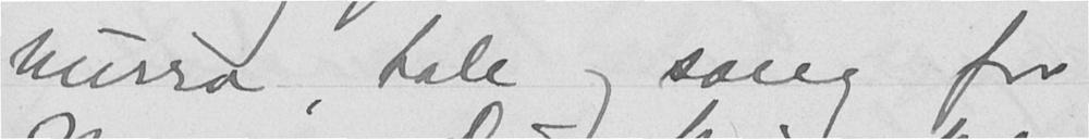hurra, tale og sang for
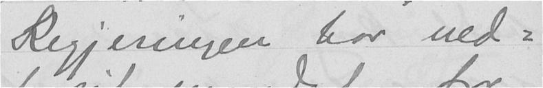Regjeringen har ned-
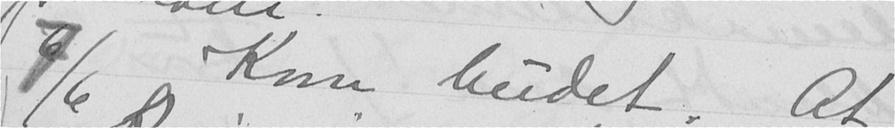7/6 Kom budet. At
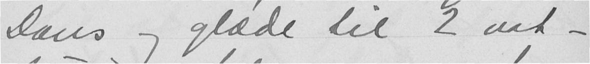Dans og glæde til 2 nat -
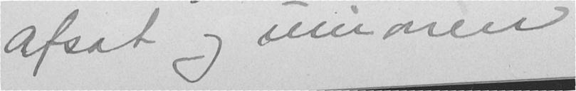afsat og unionen
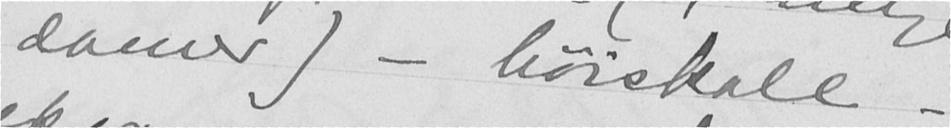damer) - høiskole-
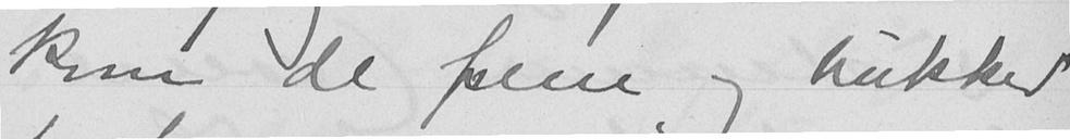kom de frem og bukked
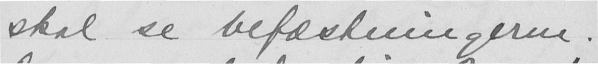skal se befæstningerne.
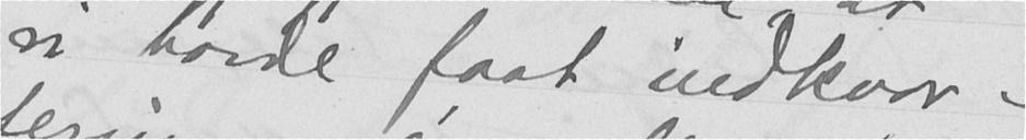vi havde faat indkvar-
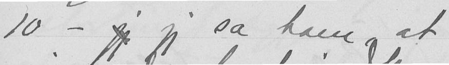10 - jeg jeg sa ham at
